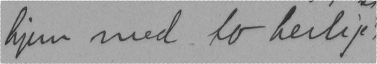hjem med to herlige
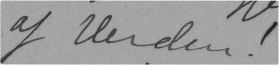af Verden!
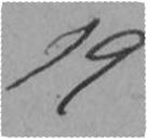19
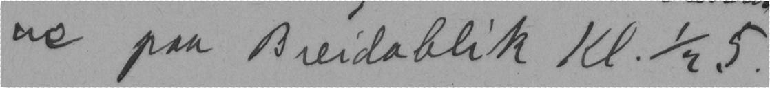ne paa Breidoblik Kl. 1/2 5.
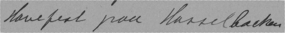Havefest paa Hasselbacken
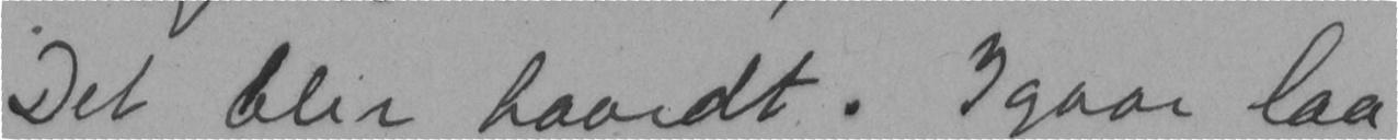Det blir haardt. Igaar laa
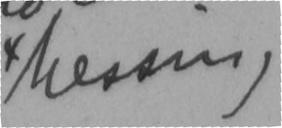Messing
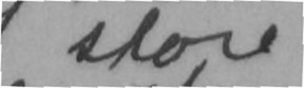store
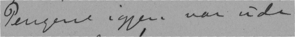Pengene igjen var ude
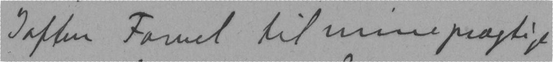I aften Farvel til mine prægtige
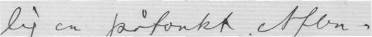lig en påtænkt Aften-
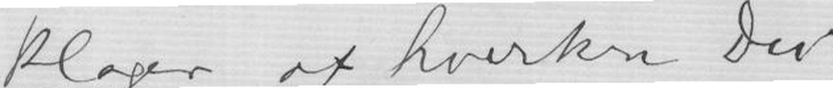klager at hverken Du
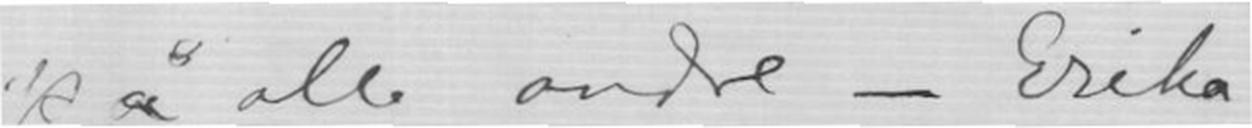på alle andre - Erika
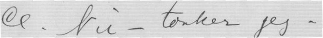ce. Nu - tænker jeg -
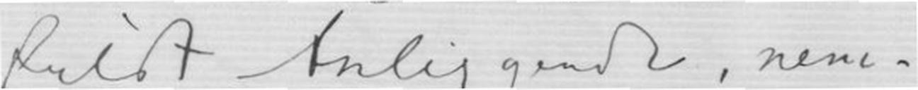fuldt Anliggende, nem-
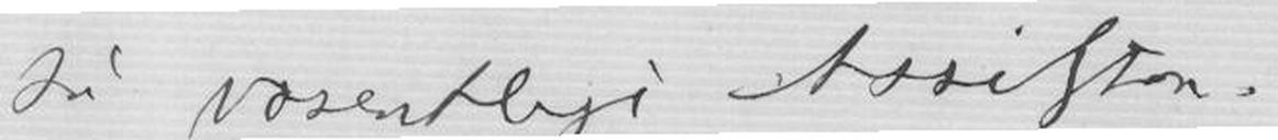så væsentlige Assistan-
